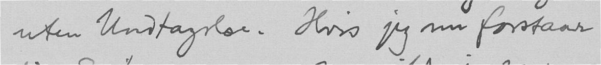uten Undtagelse. Hvis jeg nu forstaar
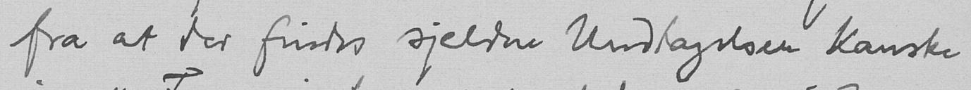fra at der findes sjeldne Undtagelser kanske
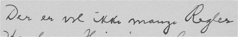Der er vel ikke mange Regler
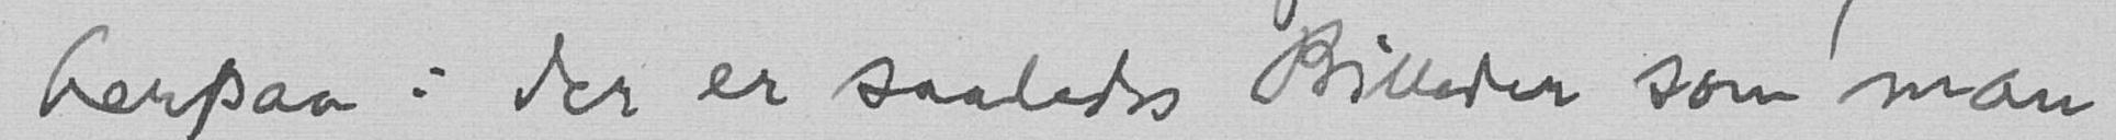herpaa: der er saaledes Billeder som man
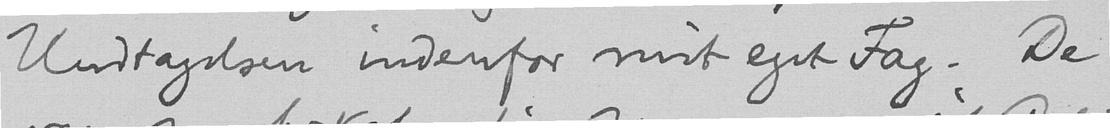Undtagelsen indenfor mit eget Fag. De
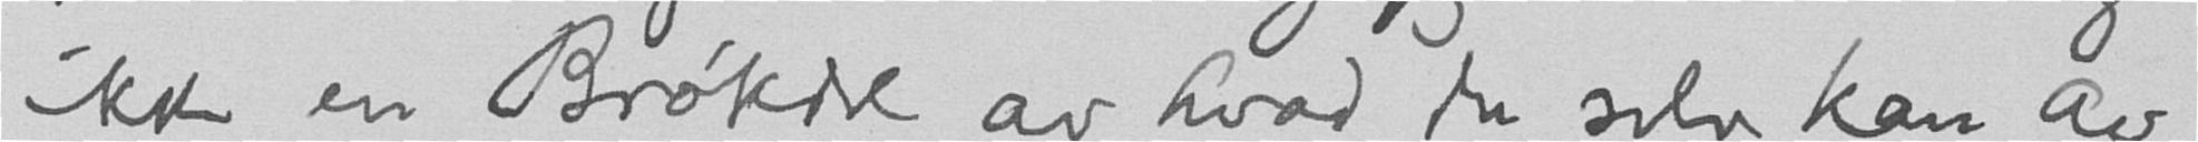ikke en Brøkdel av hvad du selv kan av
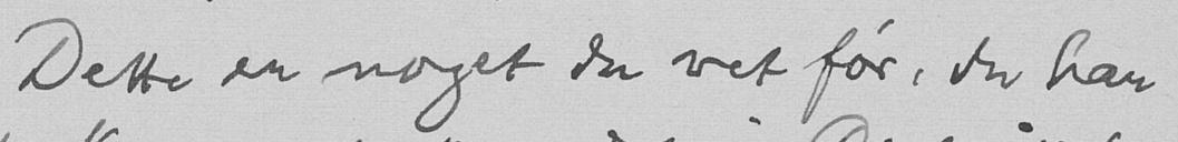Dette er noget du vet før, du har
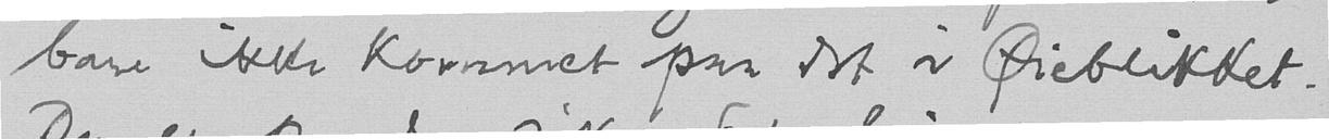bare ikke kommet paa det i Øieblikket.
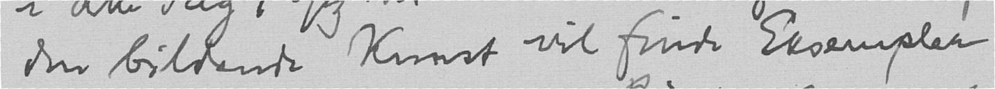den bildende Kunst vil finde Eksempler
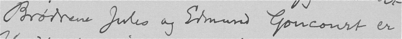Brødrene Jules og Edmund Goncourt er
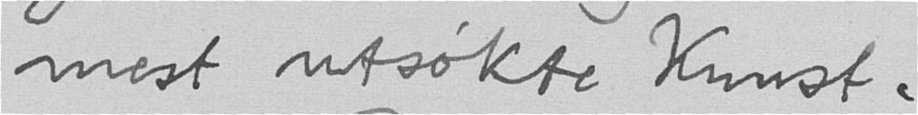mest utsøkte Kunst.
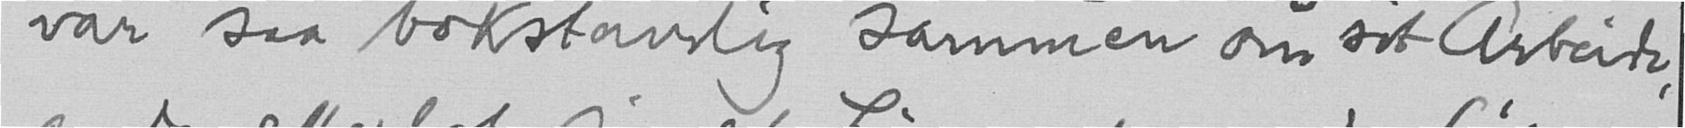var saa bokstavelig sammen om sit Arbeide,
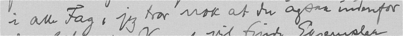i alle Fag, jeg tror nok at du ogsaa indenfor
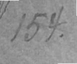154.
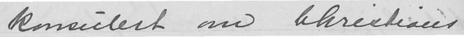konulert om Christians
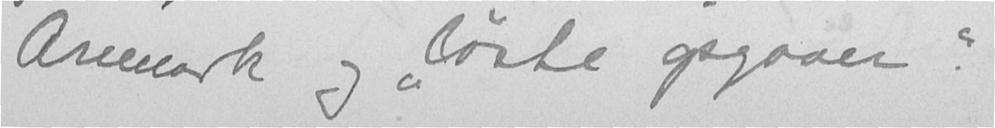Aremark og "løste opgaver".
